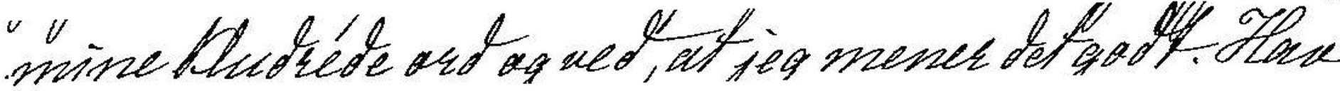mine kludrede ord og ved, at jeg mener det godt. Hav
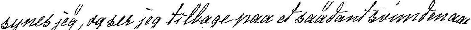synes jeg, og ser jeg tilbage paa et saadant svunden aar,
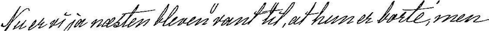Nu er vi ja næsten bleven vant til, at hun er borte, men
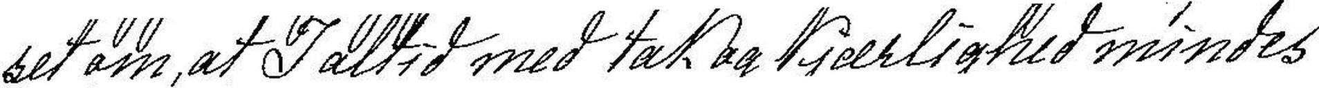set om, at I altid med tak og kjærlighed mindes
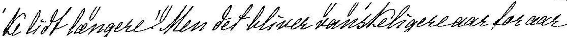ke lidt længere! Men det bliver vanskeligere aar for aar
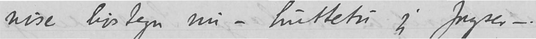vise livstegn nu – huttetu jeg fryser –.
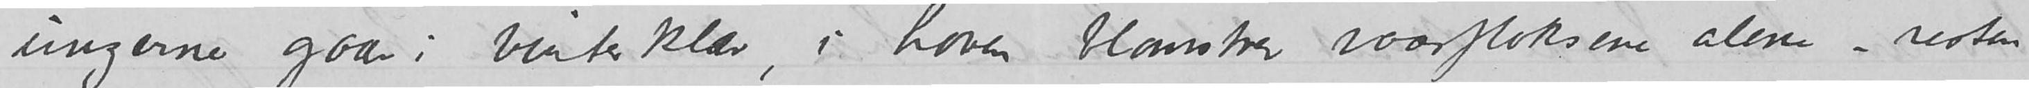ungerne gaar i vinterklær, i haven blomstrer vaarfloksene alene – resten
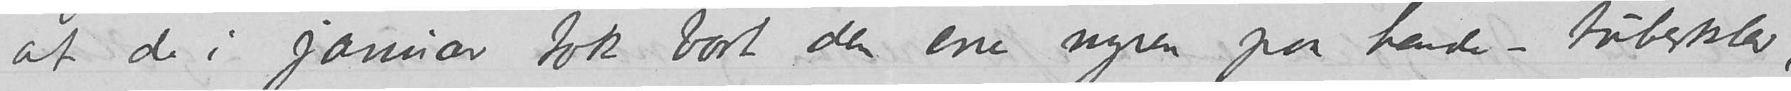at de i januar tok bort den ene nyren paa hende – tuberkler,
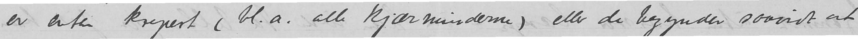er enten krepert (bl.a. alle kjærminderne) eller de begynder saavidt at
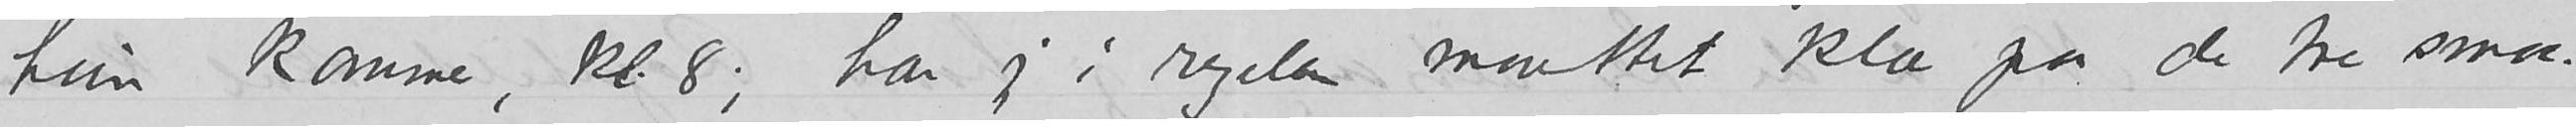hun kommer, kl. 8; har jeg i regelen maattet klæ paa de tre smaa.
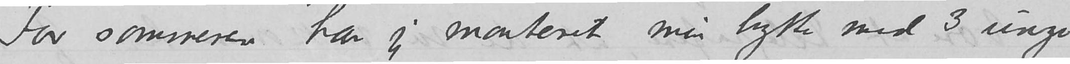For sommeren har jeg monteret min hytte med 3 unge
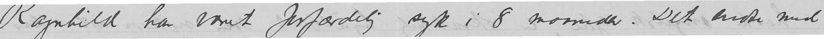Ragnhild har været forfærdelig syk i 8 maaneder. Det endte med
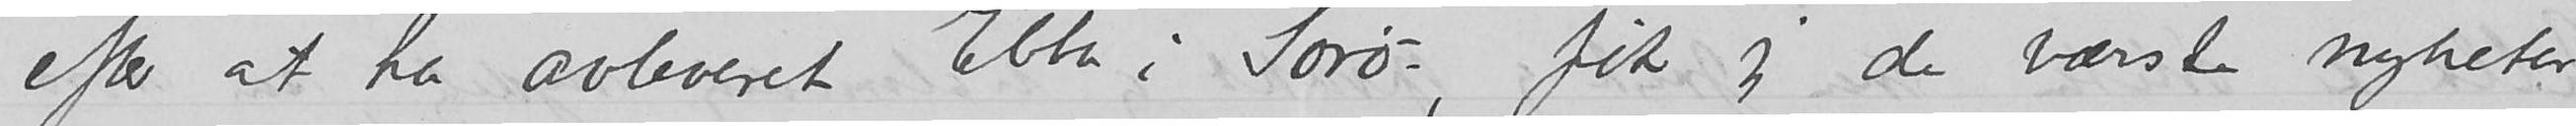efter at ha overleveret Ebba i Sorø –, fik jeg de værste nyheter
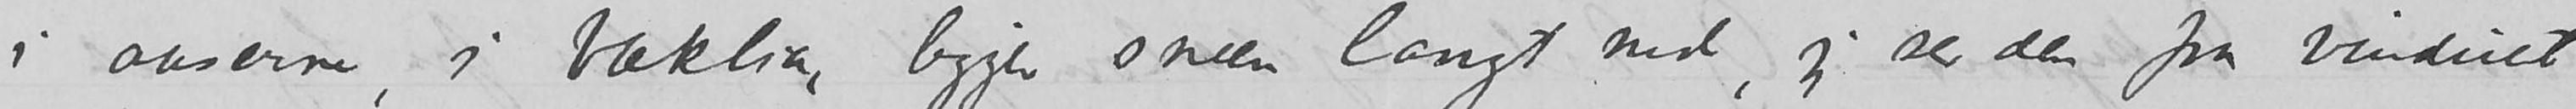i aaserne, i baklia, ligger sneen langt ned, jeg ser den fra vinduet,
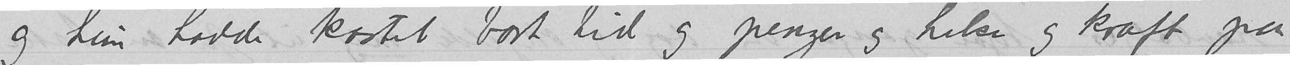og hun hadde kastet bort tid og penger og helse og kraft paa
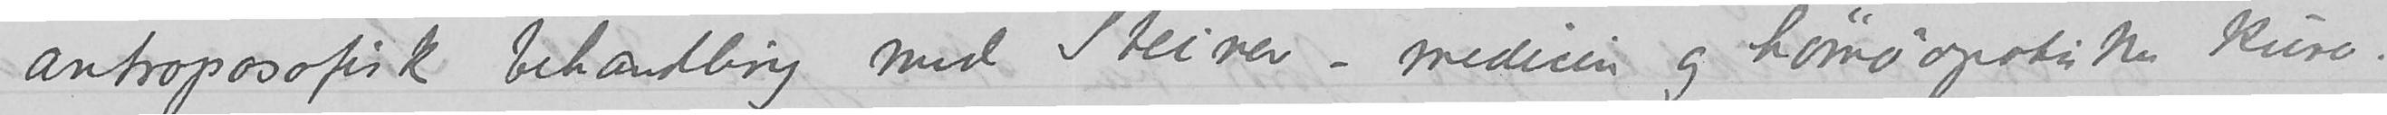antroposofisk behandling med Steiner-medicin og homøopatiske kurer.
